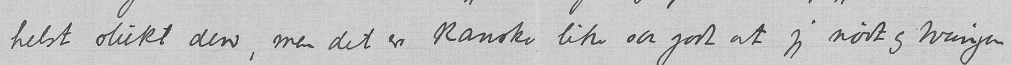helst slukt den, men det er kanske like saa godt at jeg nødt og tvungen
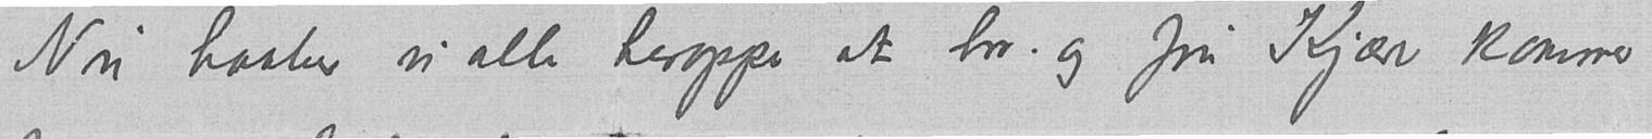Nu haaber vi alle heroppe at hr. og fru Kjær kommer
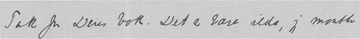Tak for Deres bok. Det er bare ilde, jeg maatte
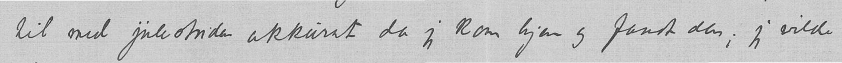til med julestriden akkurat da jeg kom hjem og fandt den; jeg vilde
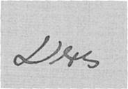Deres
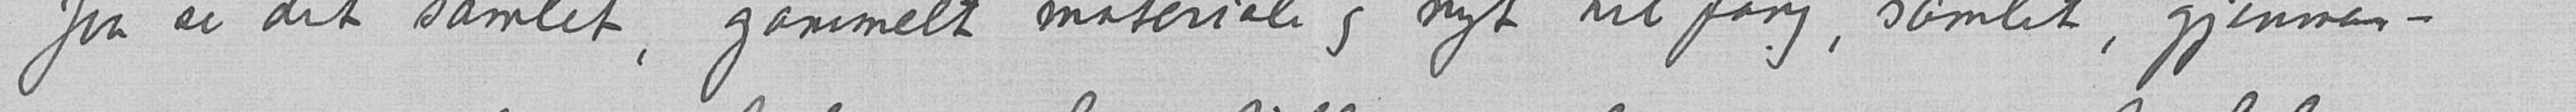faa se det samlet, gammelt materiale og nyt tilfang, samlet, gjennem-
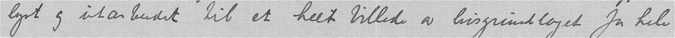lyst og utarbeidet til et helt billede av livsgrundlaget for hele
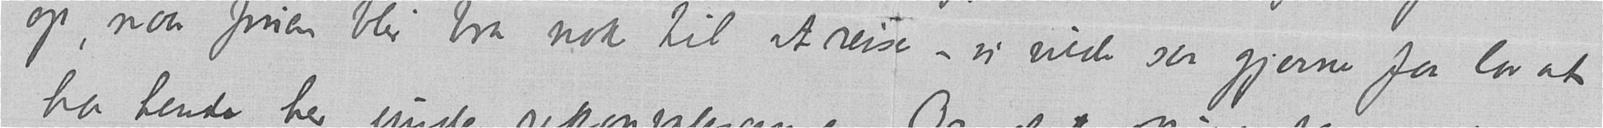op, naar fruen blir bra nok til at reise – vi vilde saa gjerne faa lov at
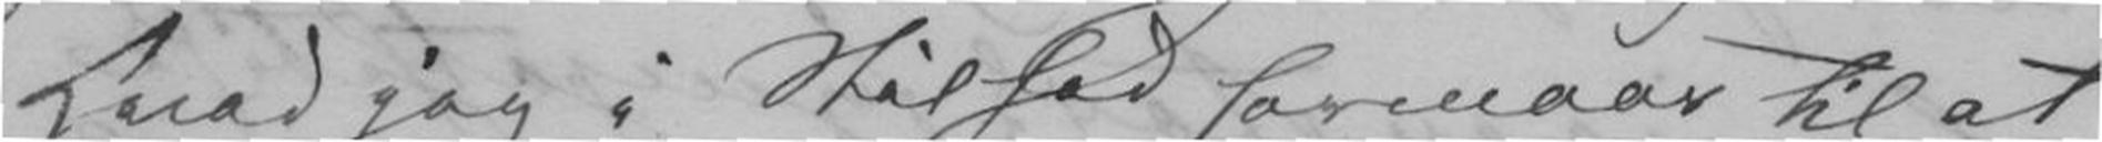Hvad jeg i stilhed formaar til at
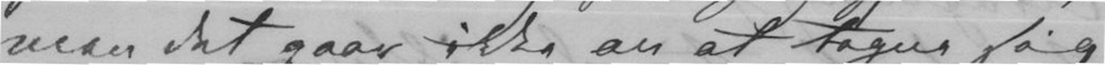men det gaar ikke an at tegne sig
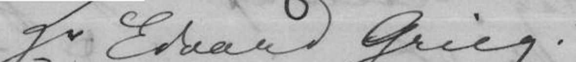Hr. Edvard Grieg.
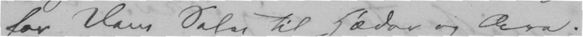for Deres Satse til Hæder og Ære.
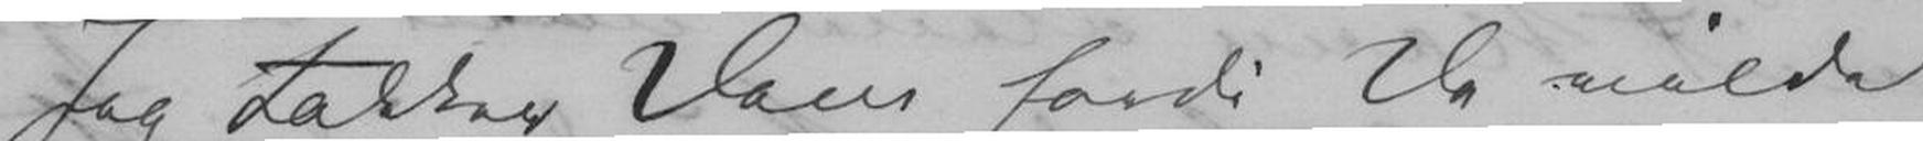Jeg takker Dem fordi De vilde
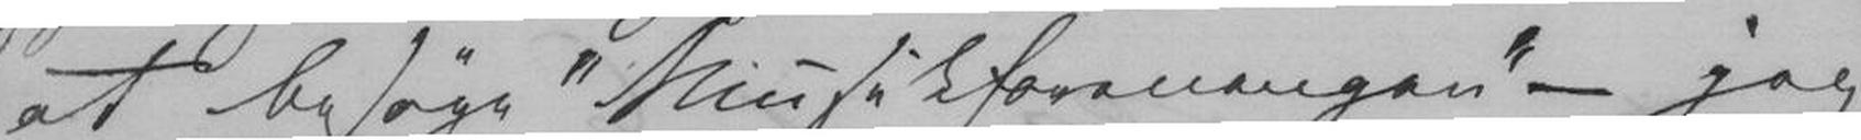at besøge "Musikforeningen" - jeg
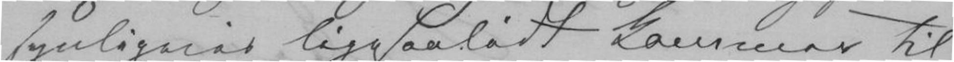synligvis ligesaalidt kommer til
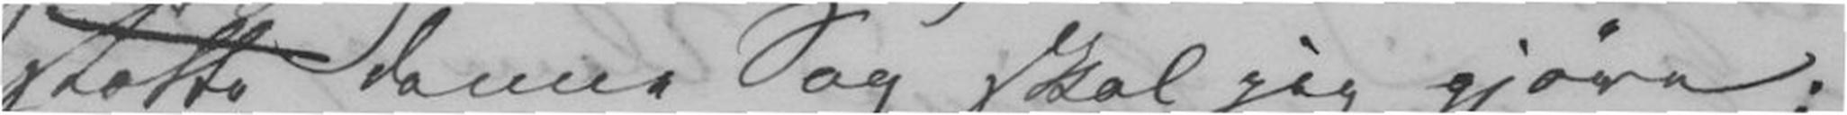støtte denne Sag skal jeg gjøre;
